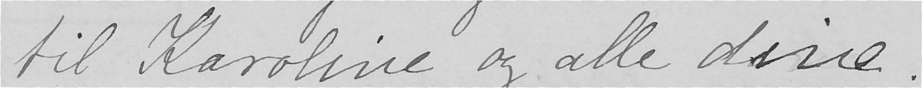til Karoline og alle dine.
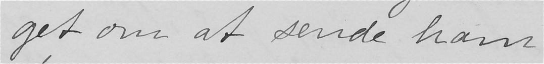get om at sende ham
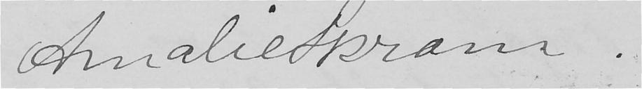Amalie Skram.
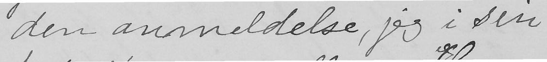den anmeldelse, jeg i sin
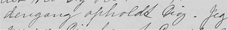dengang opholdt Dig. Jeg
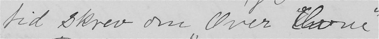tid skrev om "Over ævne"
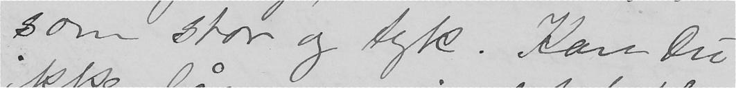som stor og tyk. Kan Du
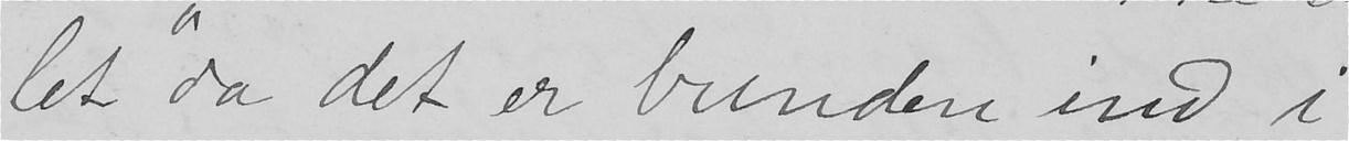let da det er bunden ind i
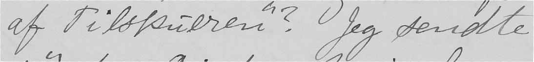af "Tilskueren"? Jeg sendte
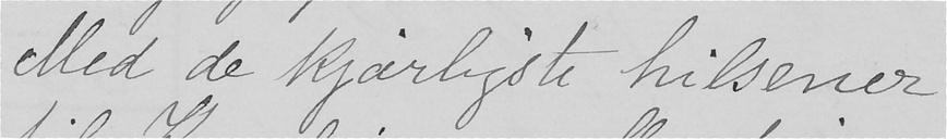Med de kjærligste hilsener
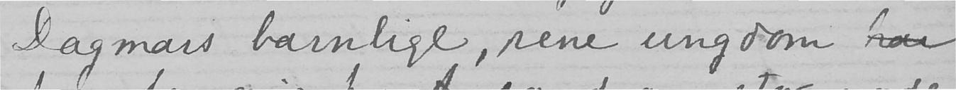Dagmars barnlige, rene ungdom har
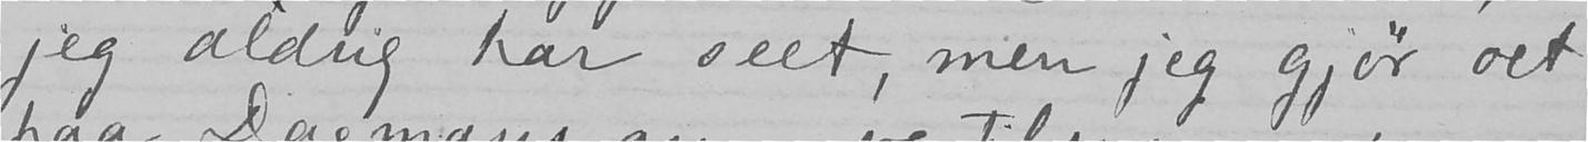jeg aldrig har seet, men jeg gjør det
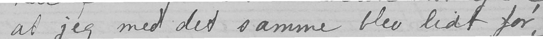at jeg med det samme blev lidt for-
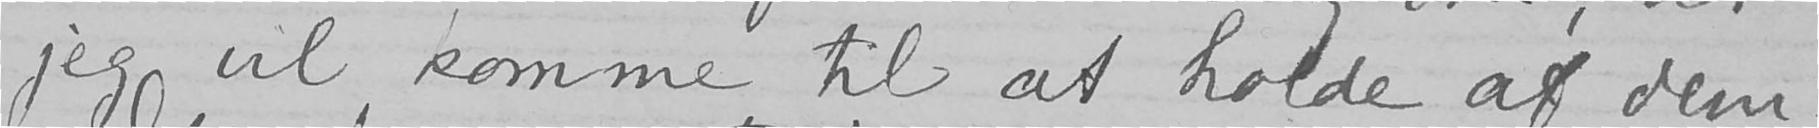jeg vil komme til at holde af dem.
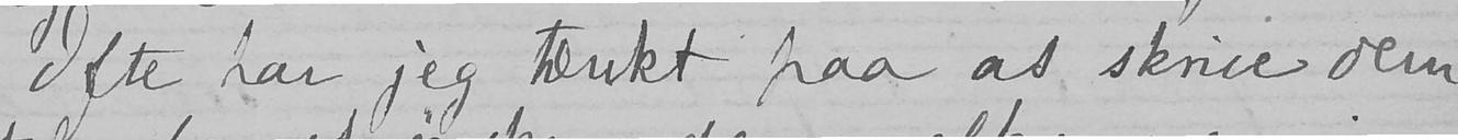Ofte har jeg tænkt paa at skrive dem
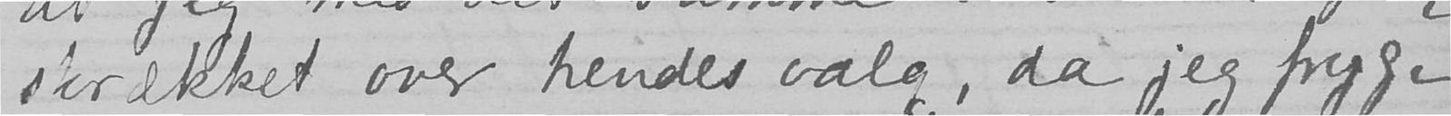skrækket over hendes valg, da jeg fryg-
